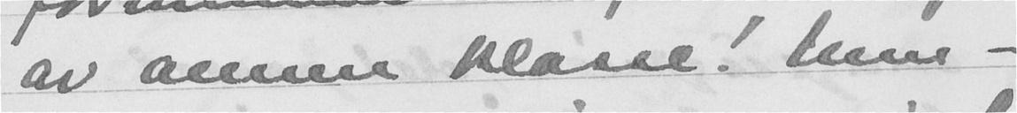av annen klasse! Kun-
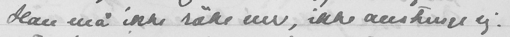Han må ikke røke mer, ikke anstrenge sig:
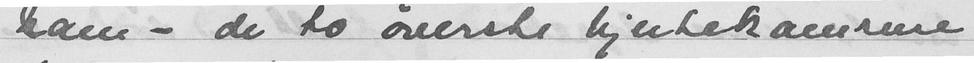ham - de to øverste hjertekamrene
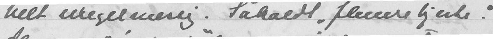helt regelmessig. Såkaldt "flimrehjerte."
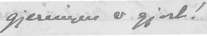gjøringen er gjort!
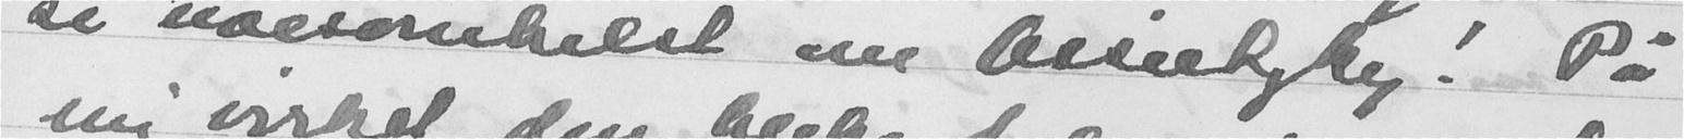si noesomhelst om Ossietzky. På
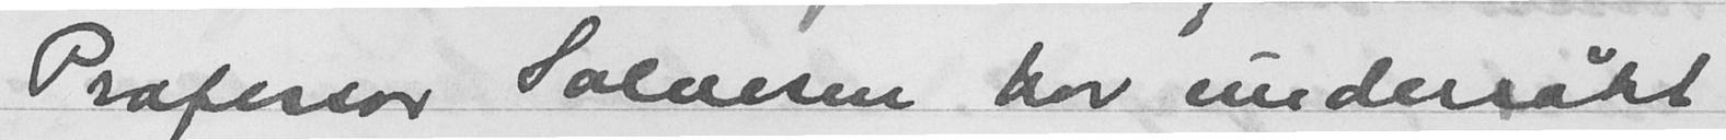Professor Solvesen har undersøkt
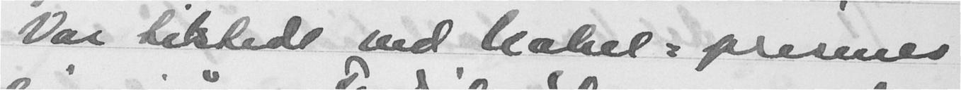Var tilstede ved nobel-prisens
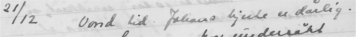21/12 Vond tid. Johans hjerte er dårlig.
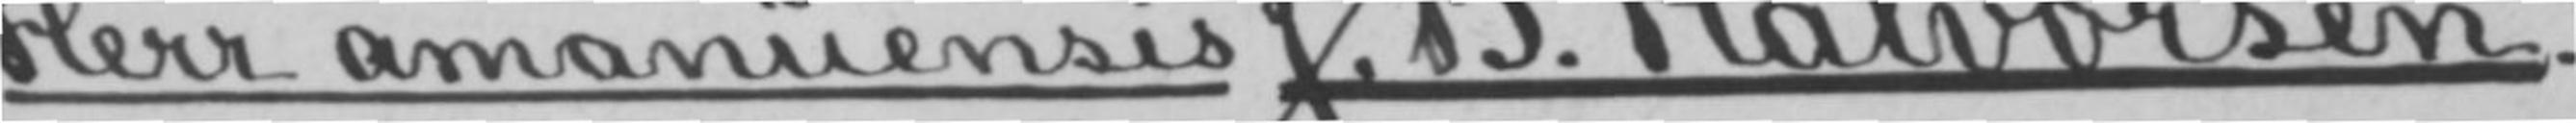Herr amanuensis J. B. Halvorsen.
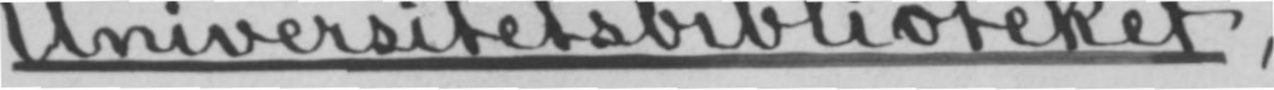Universitetsbiblioteket,
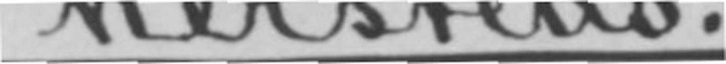hersteds.
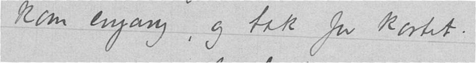kom engang, og tak for kortet.
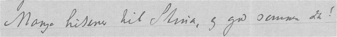Mange hilsener til Stina, og god sommer da!
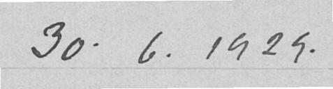30.6.1929.
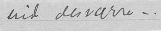ind desværre -.
